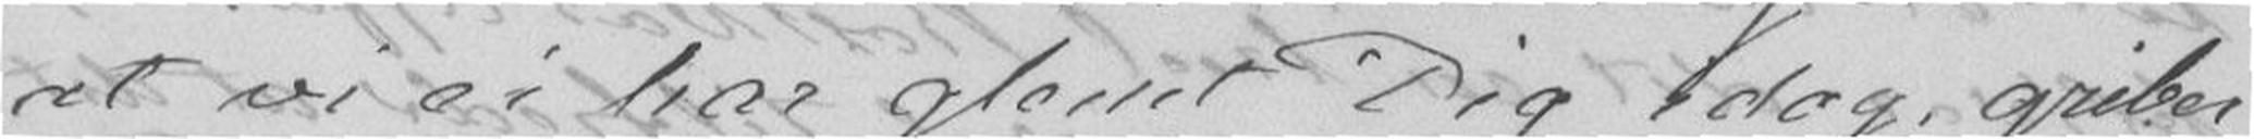at vi ei har glemt Dig idag griber
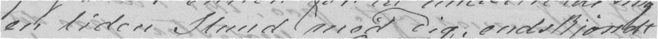en liden Stund med Dig, endskjøndt
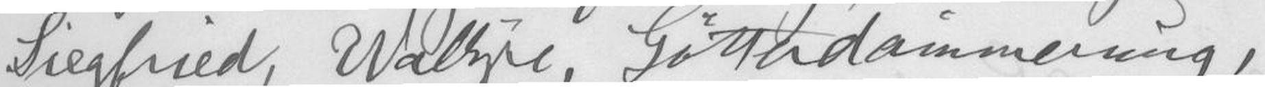Siegfried, Walkyre, Götterdämmerung,
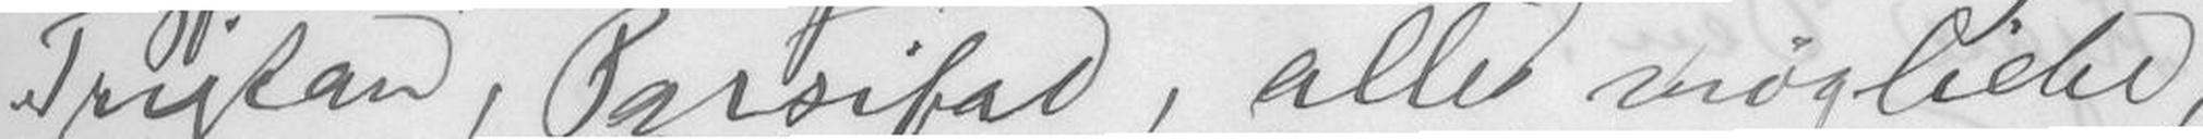Tristan, Parsifal, alles mögliche,
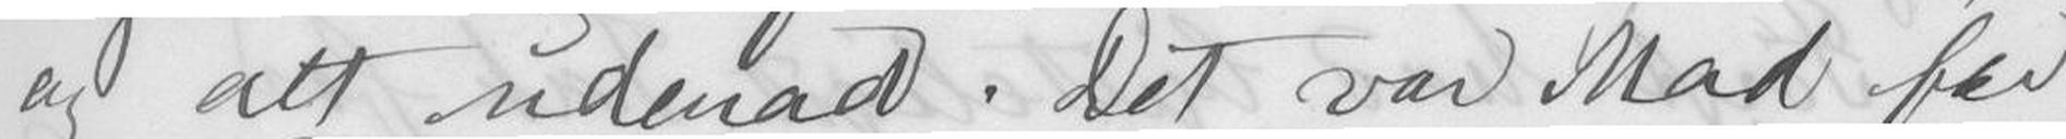og alt udenad. Det var Mad for
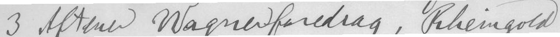3 Aftener Wagnerforedrag, Rheingold,
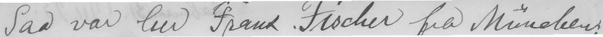Saa var her Frank Fischer fra Munchen;
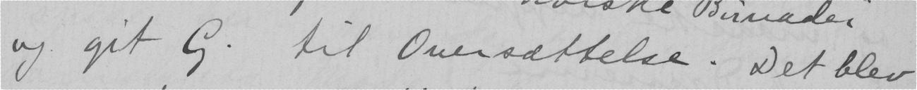og git G. til Oversættelse. Det blev
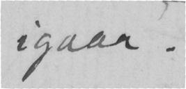i gaar.
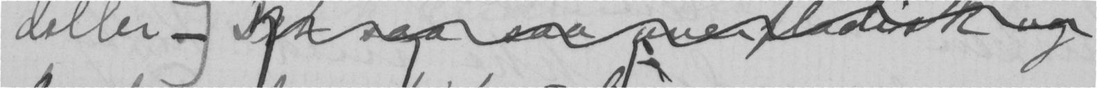deller -
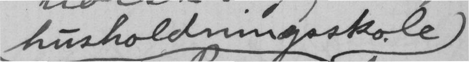husholdningsskole
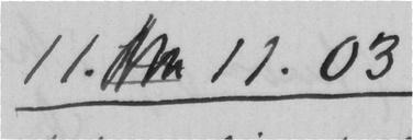11.  11.03
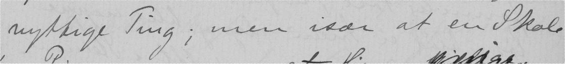nyttige Ting; men især at en Skole
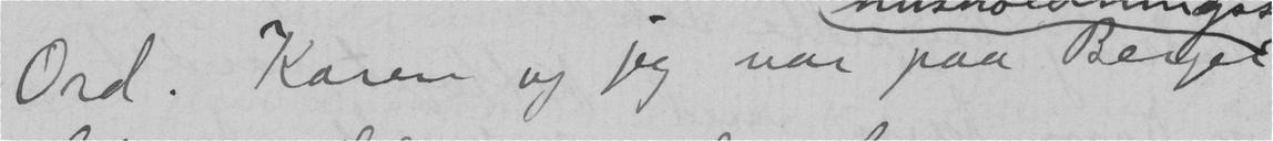Ord. Karen og jeg var paa Beyer
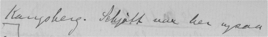Kongsberg. Schjøtt var her ogsaa
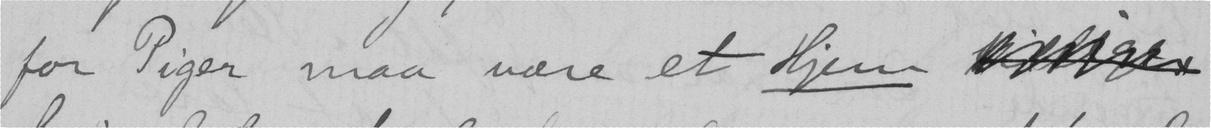for Piger maa være et Hjem
